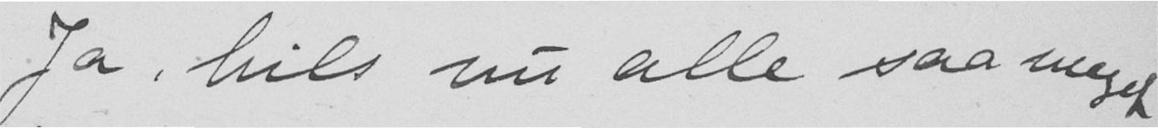Ja, hils nu alle saa meget
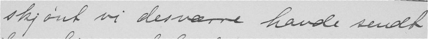skjønt vi desværre havde sendt
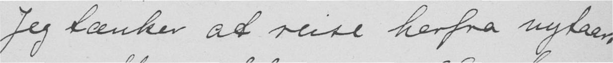Jeg tænker at reise herfra nytaars-
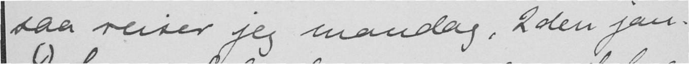saa reiser jeg mandag, 2den jan.
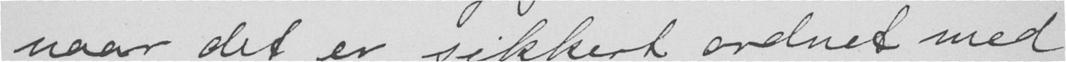naar det er sikkert ordnet med
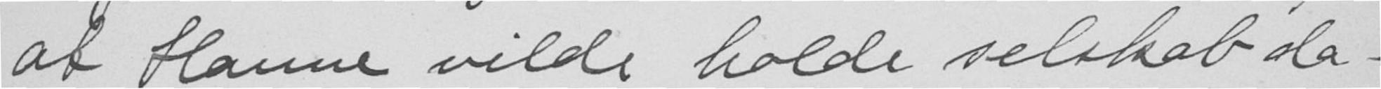at Hanne vilde holde selskab da -
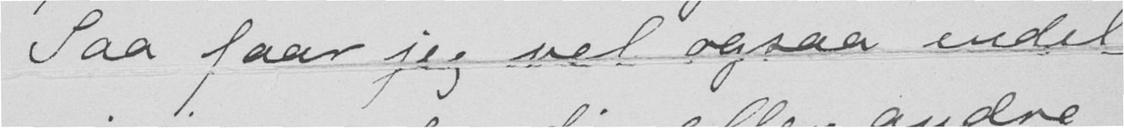Saa faar jeg vel ogsaa endel
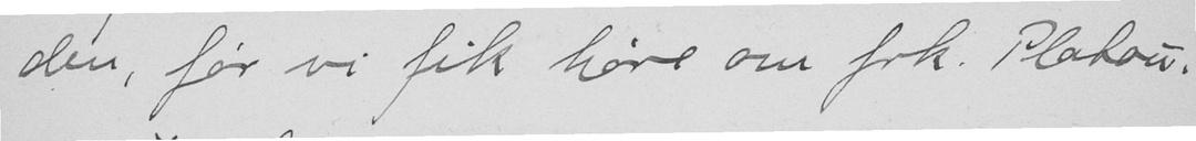den, før vi fik høre om frk. Platou.
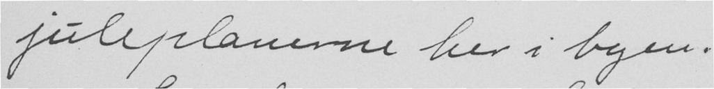juleplanerne her i byen.
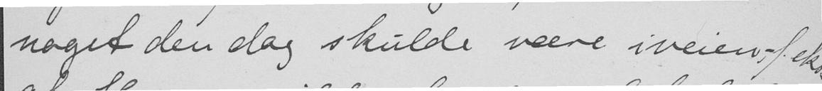noget den dag skulde være iveien, - f.eks
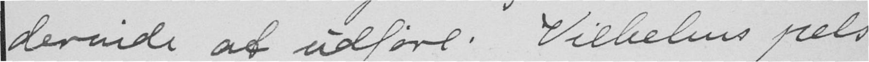dernide at udføre. Vilhelms pels
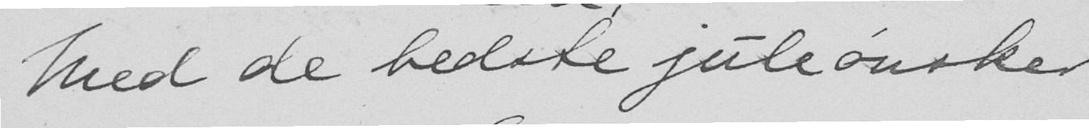Med de bedste juleønsker
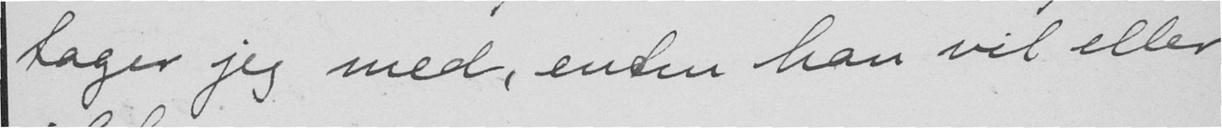tager jeg med, enten han vil eller
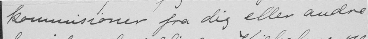kommisioner fra dig eller andre
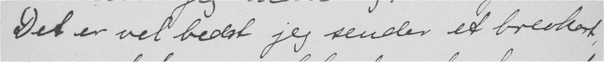Det er vel bedst jeg sender et brevkort,
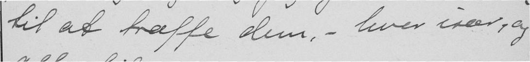til at træffe dem, - hver især, og
